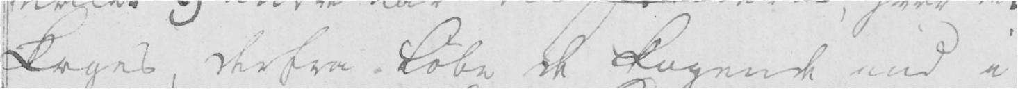koges, derfra løbe de kogende ned i
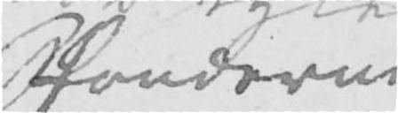Fonderne
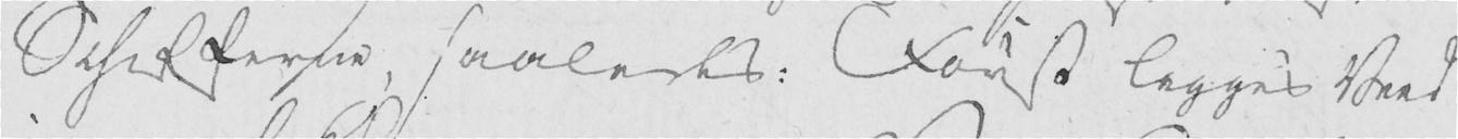Schifferne saaledes: Først legges Veed
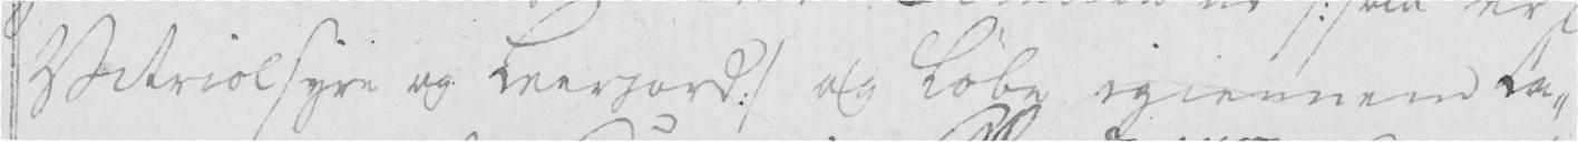Vitriolsyre og Leerjord :/ og løbe igiennem Ca-
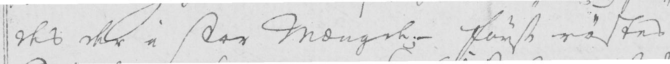des der i stor Mængde; - først røstes
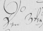Den 3die
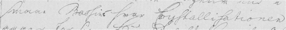smaae Bassins hvor Crystallisationen
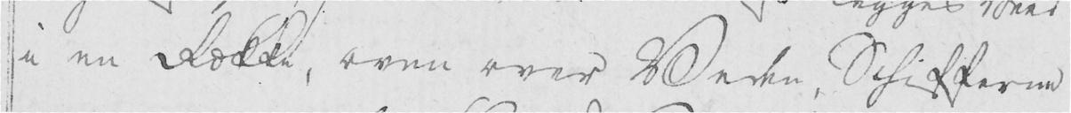i en Række, oven over Veden, Schifferne
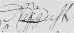August
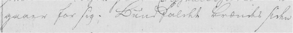gaaer for sig; Bundfaldet brændes siden
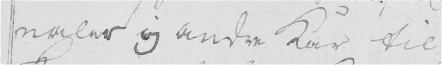naler og andre Kar til
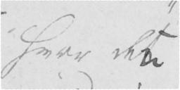hvor det
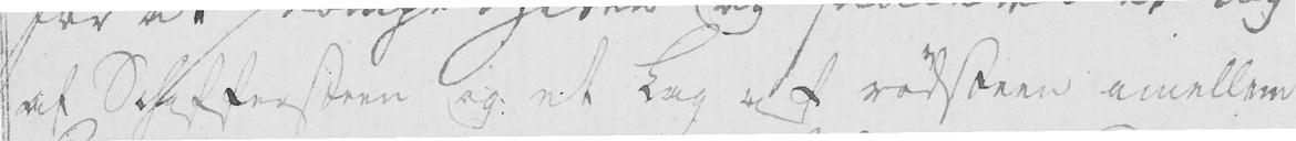af Schiffersteen og et Lag af rødsteen imellem
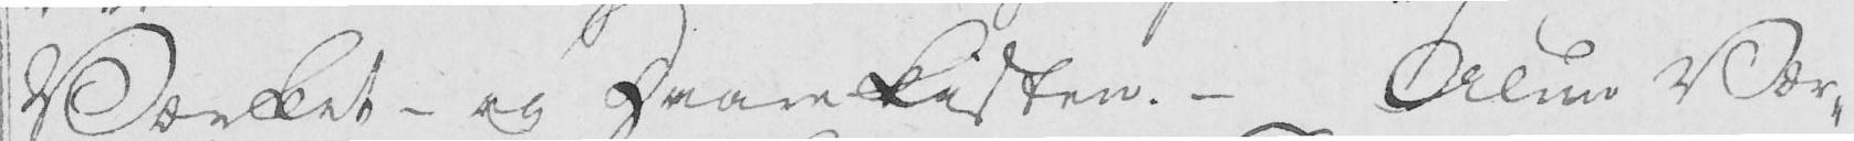Værket - og Daarekisten. - Alun Vær-
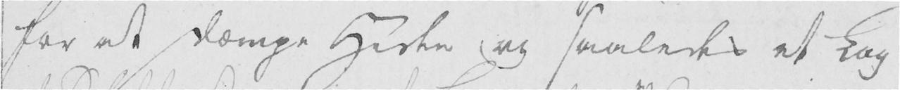for at dæmpe Heden og saaledes et Lag
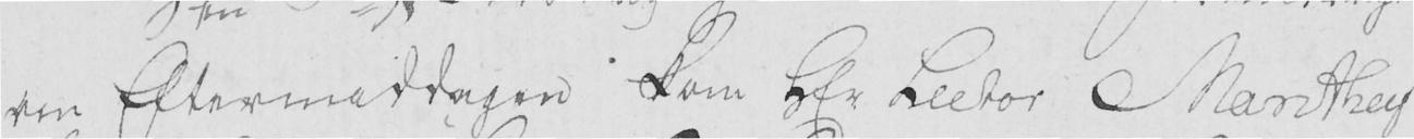om Eftermiddagen kom Hr Lector Manthey
